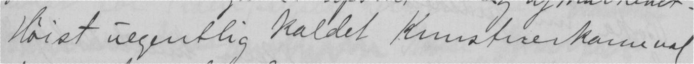Høist uegentlig kaldet Kunstnerkarneval
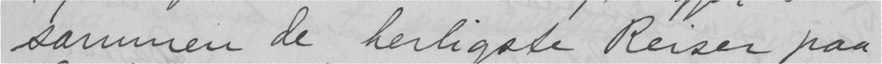sammen de herligste Reiser paa
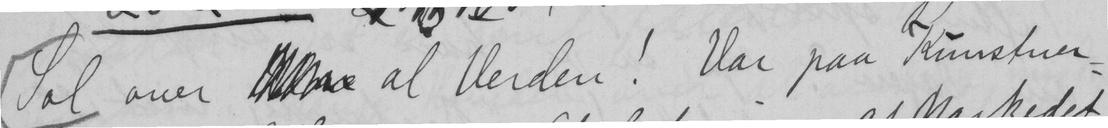Sol over  al verden! Var paa Kunstner-
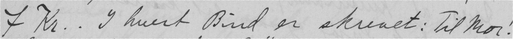7 Kr.. I hvert Bind er skrevet: Til Mor!
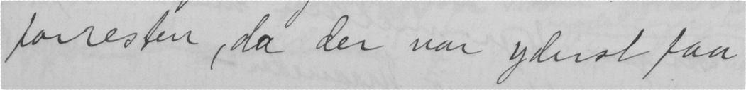forresten, da der var yderst faa
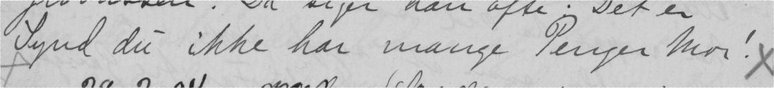synd du ikke har mange Penger Mor! x
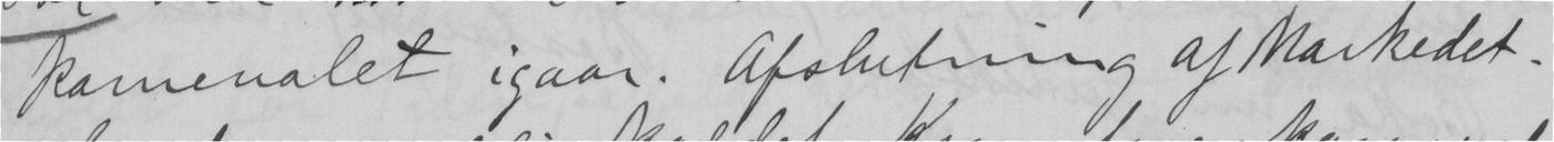karnevalet igaar. Atslutning af Markedet.
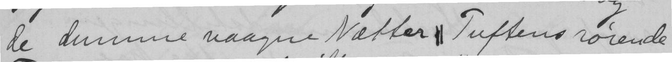de dumme vaagne Nætter, Tuftens rørende
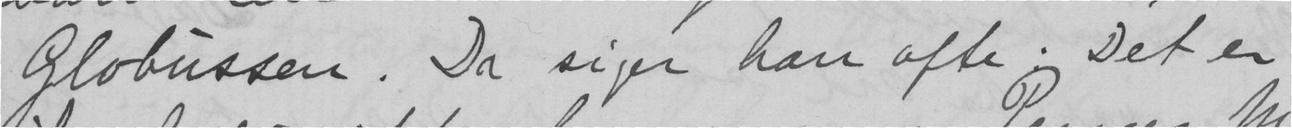Globussen. Da siger han ofte: Det er
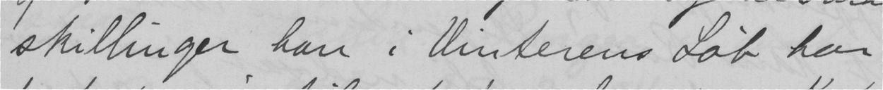skillinger han i Vinterens Løb har
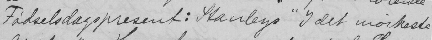Fødselsdagspresent: Stanleys "I det mørkeste
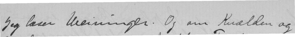Jeg læser Weininger. Og om Kvælden og
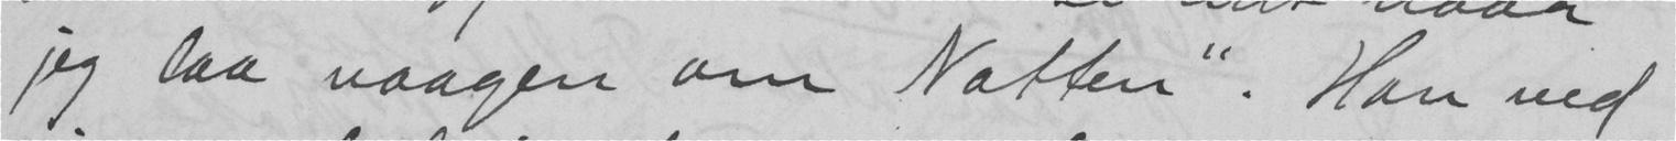jeg laa vaagen om Natten". Han ved
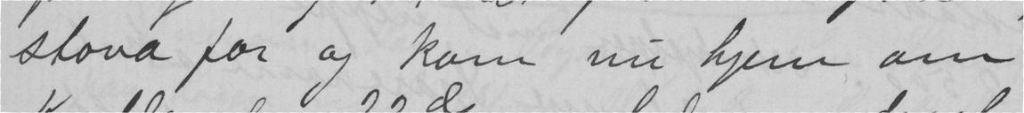stova for og kom nu hjem om
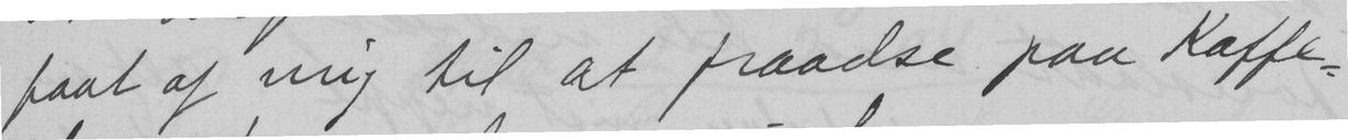faat af mig til at fraadse paa Kaffe-
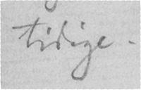tidige.
